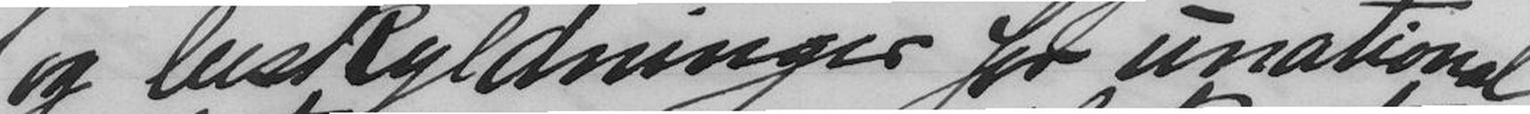og beskyldninger for unational
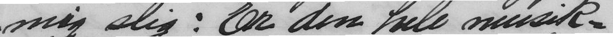mig slig: Er den hele musik-
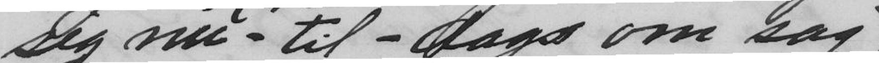sig nu - til - dags om sag
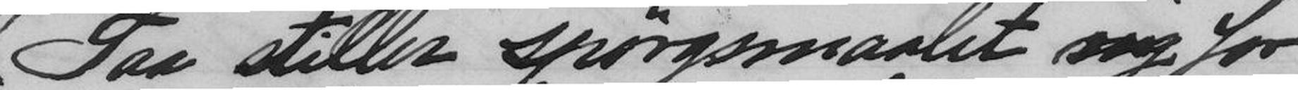Saa stiller spørgsmaalet sig for
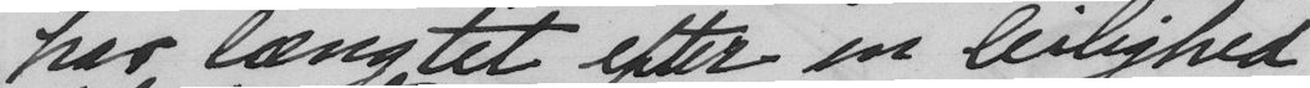har længtet efter en leilighed
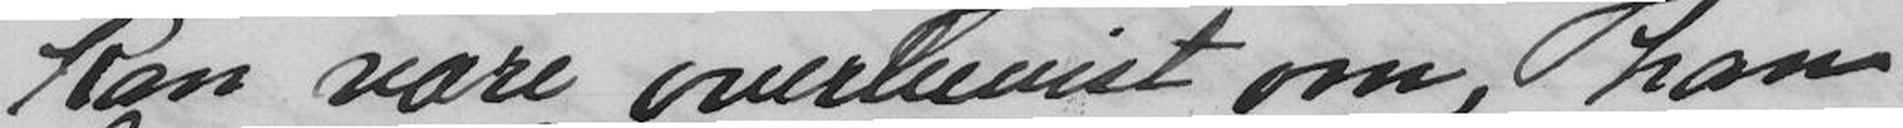kan være overbevist om, han
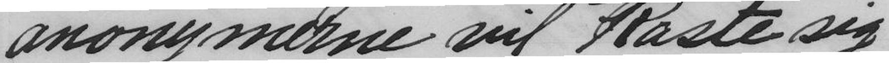anonymerne vil kaste sig
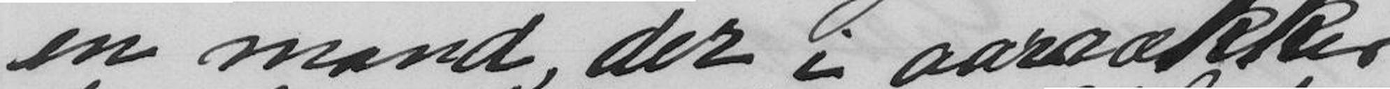en mand, der i aarrækker
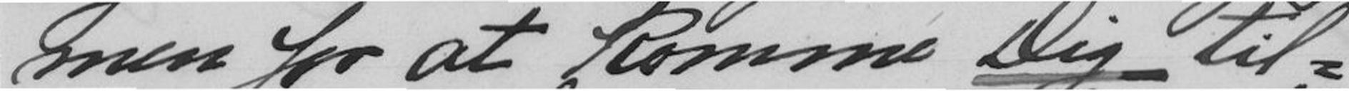men for at komme Dig til-
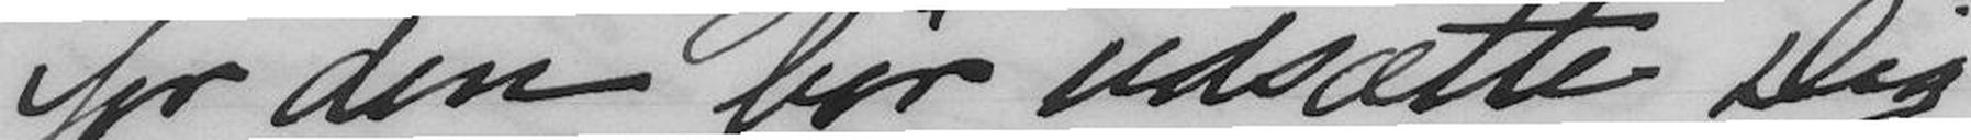for den bør udsætte Dig
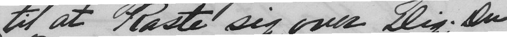til at kaste sig over Dig. Du
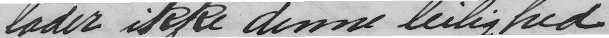lader ikke denne leilighed
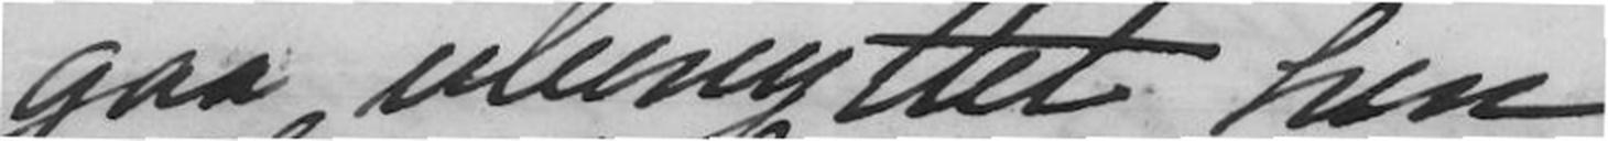gaa ubenyttet hen.
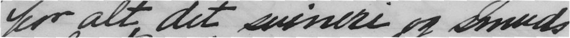for alt det svineri og smuds
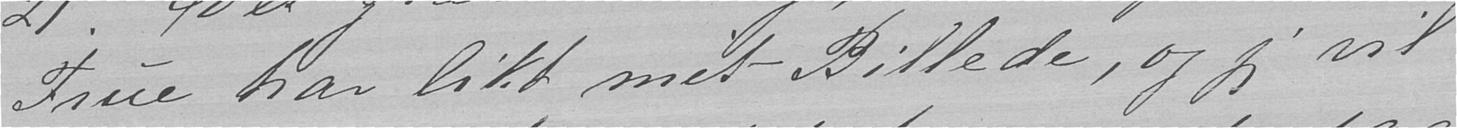Frue har likt mit Billede, og jeg vil
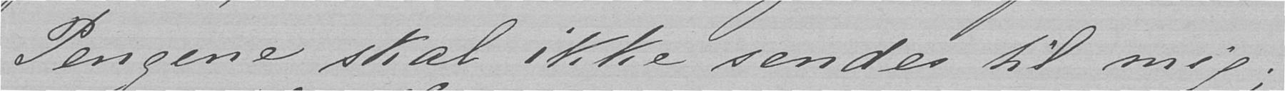Pengene skal ikke sendes til mig;
